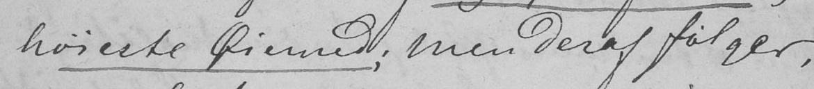høieste Øiemed; men deraf følger,
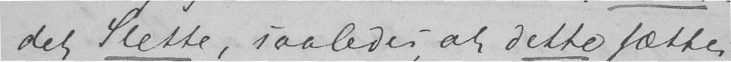det Slette, saaledes at dette sættes
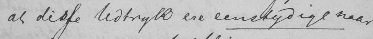at disse Udtryk ere eenstydige naar
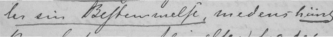ler sin Bestemmelse, medens hiin
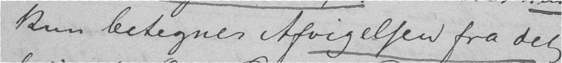kun betegner Afvigelsen fra det
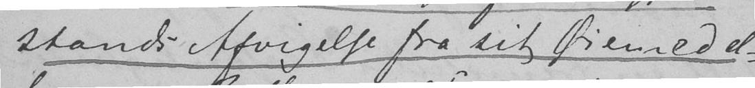stands Afvigelse fra sit Øiemed el-
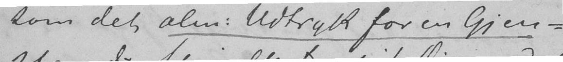som det alm: Udtryk for en Gjen-
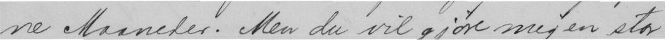ne Maaneder. Men du vil gjøre mig en stor
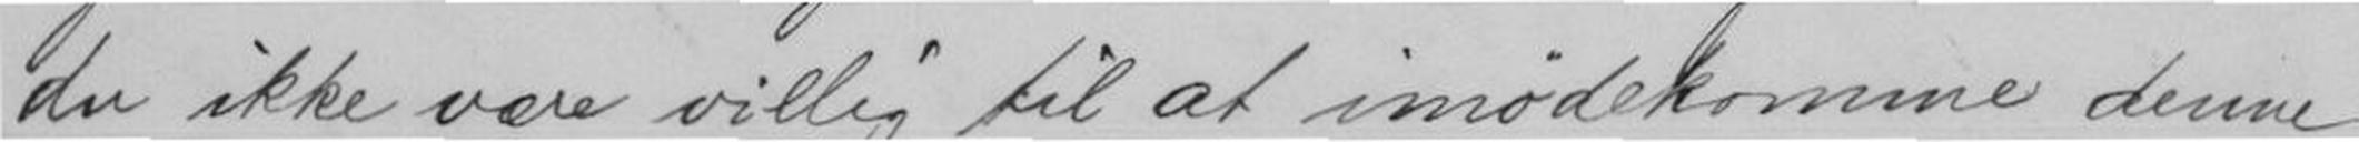du ikke være villig til at imødekomme denne
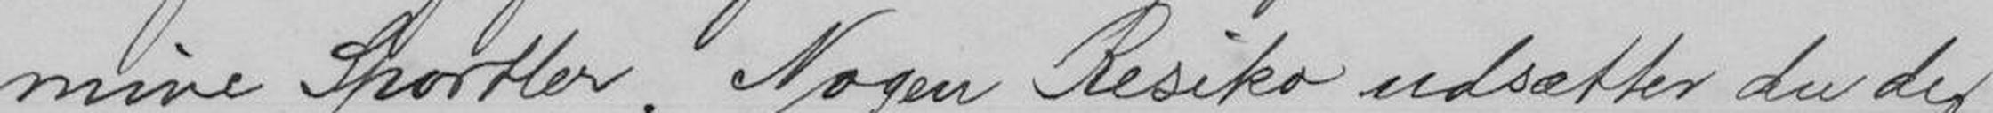mine Sportler. Nogen Risiko udsætter du dig
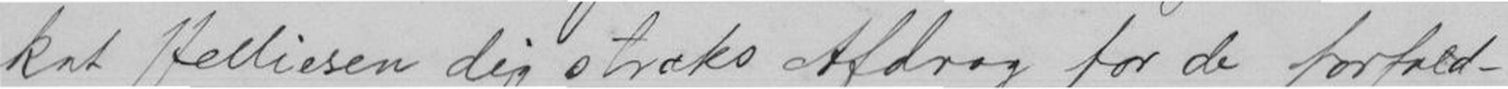kat Helliesen dig straks Afdrag for de forfald-
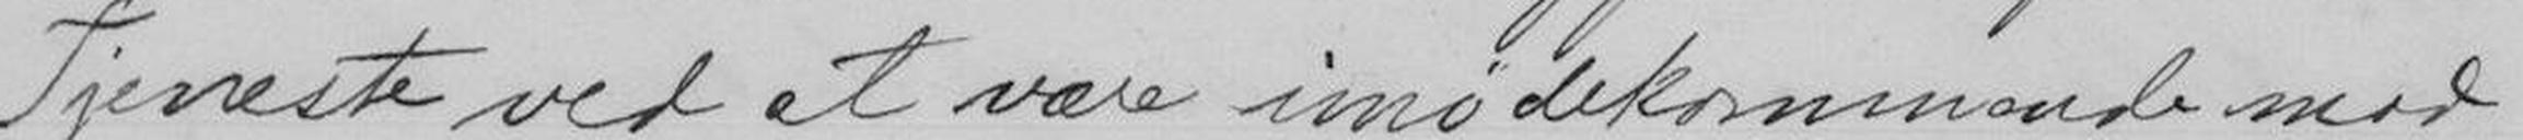Tjeneste ved at være imødekommende mod
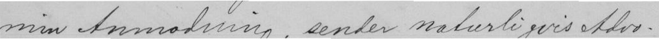min Anmodning, sender naturligvis Advo-
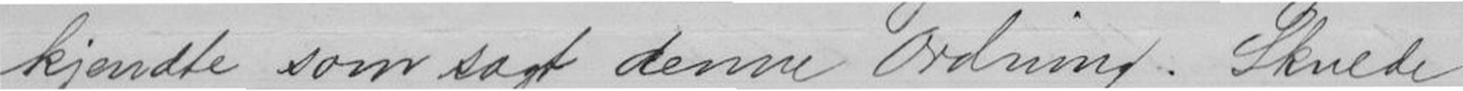kjendte som sagt denne Ordning. Skulde
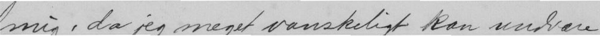mig, da jeg meget vanskeligt kan undvære
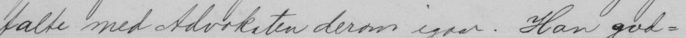talte med Advokaten derom igaar. Han god-
